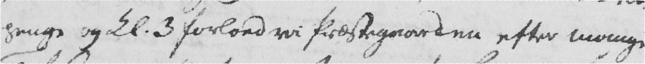penge og Kl. 3 forloed vi Præstegaarden efter mange
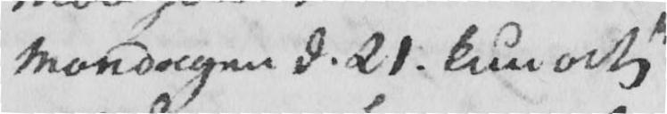Mandagen d. 21. kun at
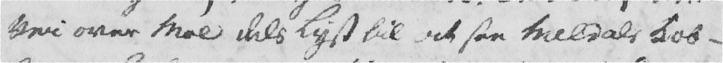Vei over Moe dels lyst til at see Meldals Kob-
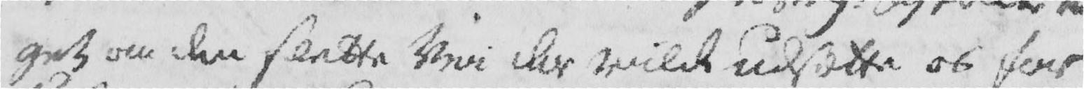get om den slette Vei der vilde udsætte os for
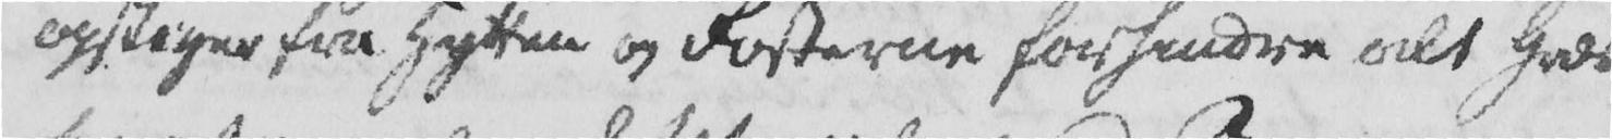opstiger fra Hytten og Rosterne forhindre alt Græs
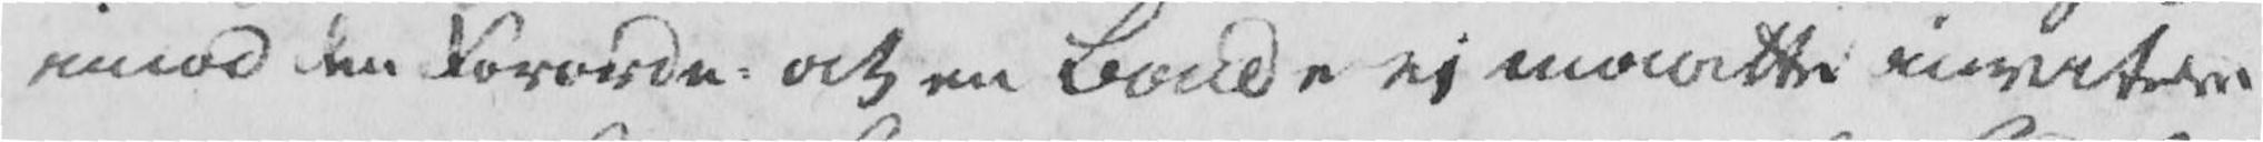imod den Forordn. at en Bonde ej maatte invitere
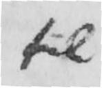til
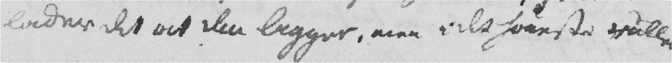lader det at den ligger, men i det høieste ruller
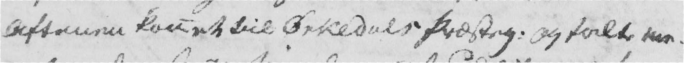Aftenen komet til Ørkedals Præsteg. og talte me-
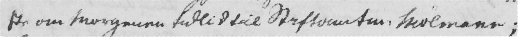ste om Morgenen tidlig til Stiftamtm. Mølmann;
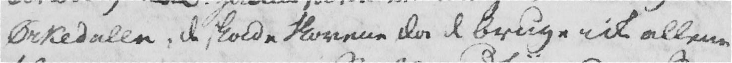Ørkedalen, de skade Skovene da de bruge icke allene
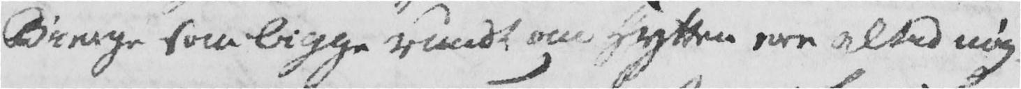Bierge som ligge rundt om Hytten ere altid nøg-
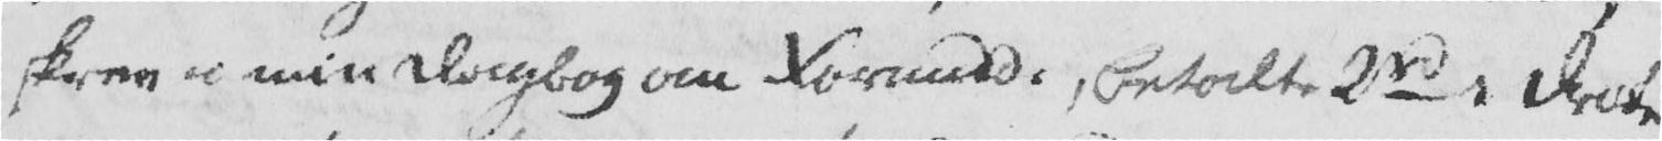skrev i min Dagbog om Formidd., betalte 2 rd i Dricke-
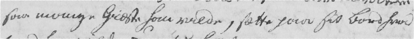saa mange Giæste han vilde, sætte paa sit Bord hvad
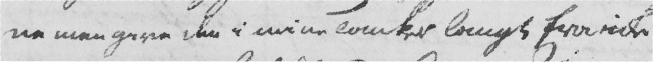ne men give dem i mine Tanker langt fra icke
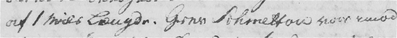af 1 Mils Længde. Grev Schmettou var imod
